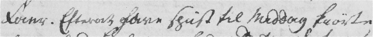Faer. Efterat have spist til Middag kiørte
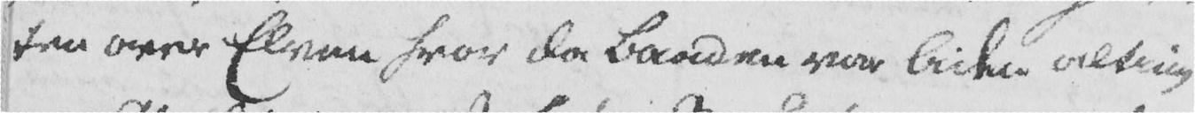ten over Elven hvor da Baaden var liden alting
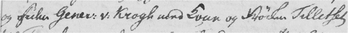og siden Gener. v. Krogh med Kone og Frøken Telletset
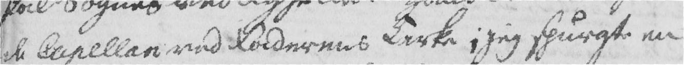de Capellan ved Faderens Kirke; jeg spurgte en
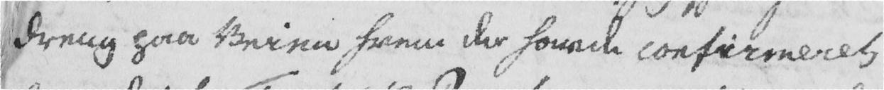Dreng paa Veien hvem der havde confirmeret
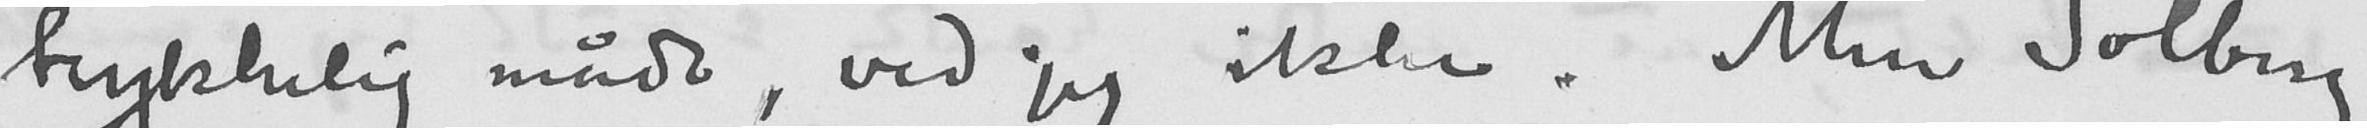trykkelig måde, ved jeg ikke. Men Solberg
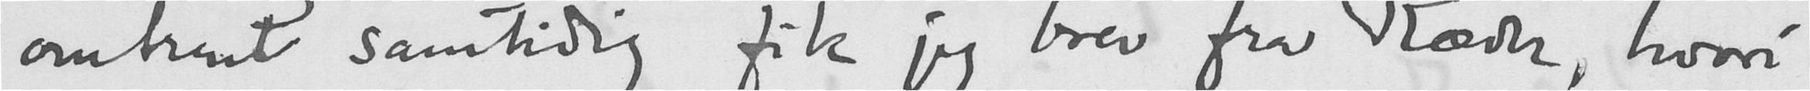omtrent samtidig fik jeg brev fra Ræder, hvori
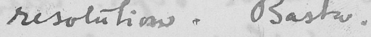resolution. Basta.
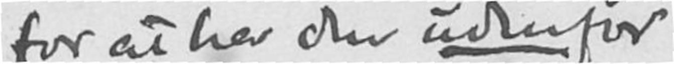for at ha den udenfor
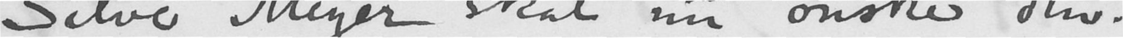Selve Meyer skal nu ønske den.
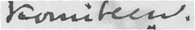komiteen.
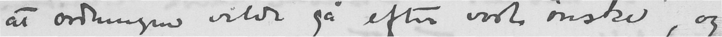at ordningen vilde gå efter vort ønske", og
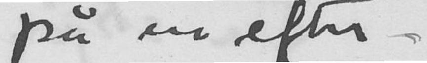på en efter -
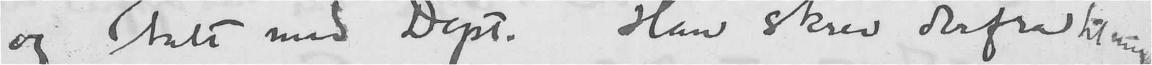og talt med Dept. Han skrev derfra til mig
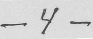- 4 -
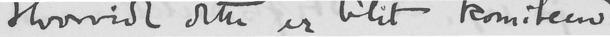Hvorvidt dette er blit komiteen
